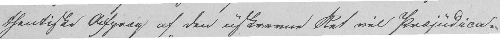thentiske Afpræg af den uskrevene Ret vil Præjudica-
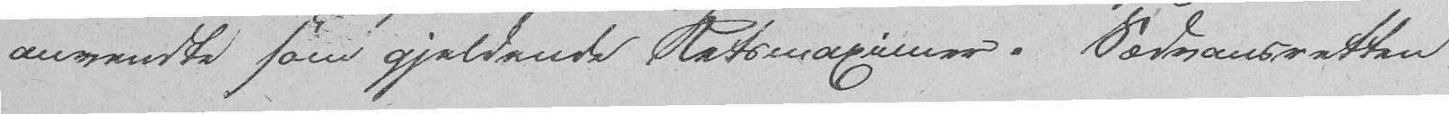anvendte som gjeldende Retsmaximer. Sædvansretten
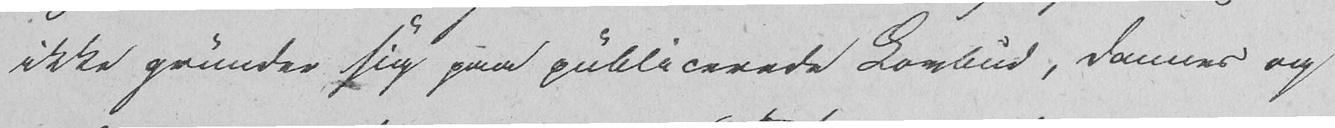ikke grunder sig paa publicerede Lovbud, dannes og
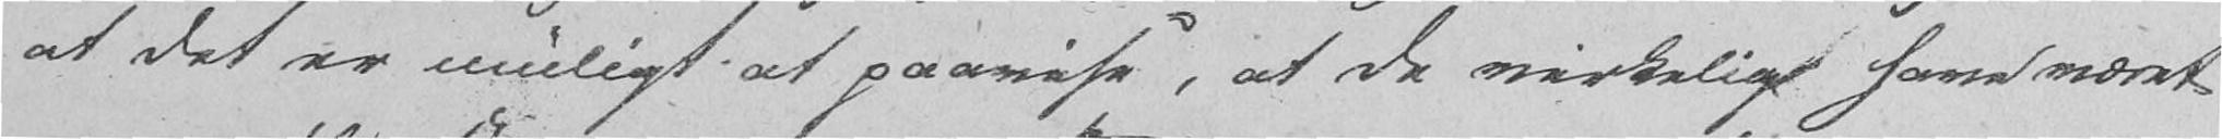at det er muligt at paavise, at de virkelig have været
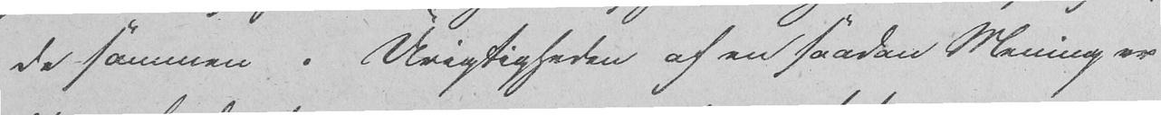de sammen. Urigtigheden af en saadan Mening er
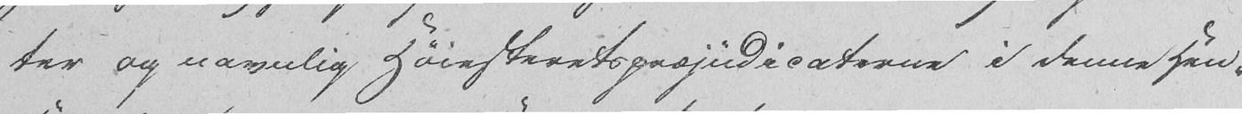ter og navnlig Høiesteretspræjudicaterne i denne Hen-
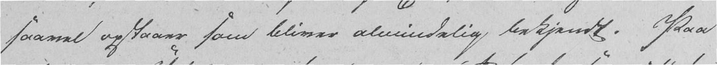saavel opstaaer som bliver almindelig bekjendt. Paa
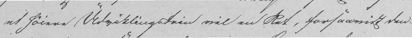et høiere Udviklingstrin vil en Ret, forsaavidt den
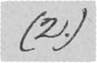(2.)
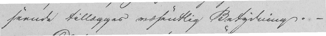seende tillægges væsentlig Betydning. -
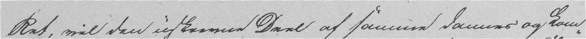Ret, vil den uskrevne Deel af samme dannes og kom-
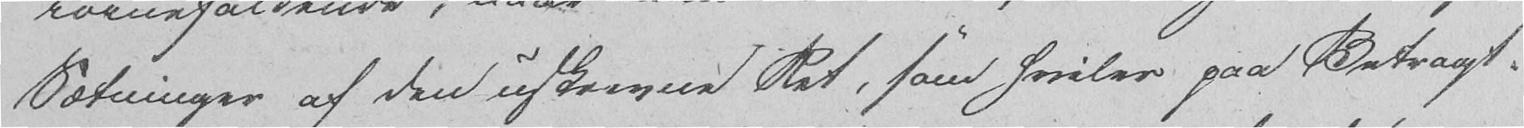Sætninger af den uskrevne Ret, som hviler paa Betragt-
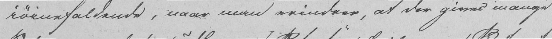iøinefaldende, naar man erindrer, at der gives mange
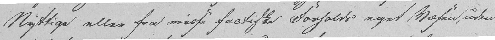Nyttige eller fra visse factiske Forholds eget Væsen, uden
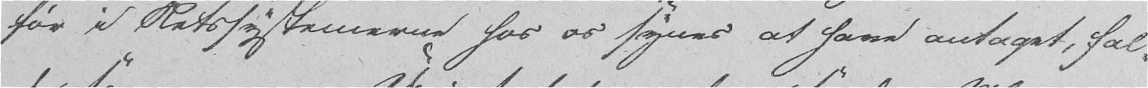før i Retssystemerne hos os synes at have antaget, fal-
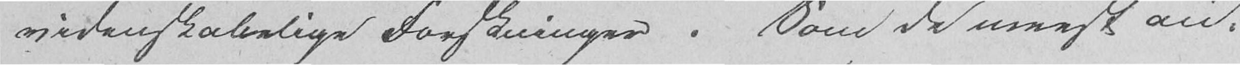videnskabelige Forskninger. Som de meest au-
